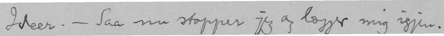Ideer. - Saa nu stopper jeg og lægger mig igjen.
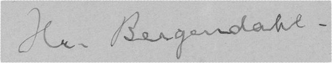Hr. Bergendahl.
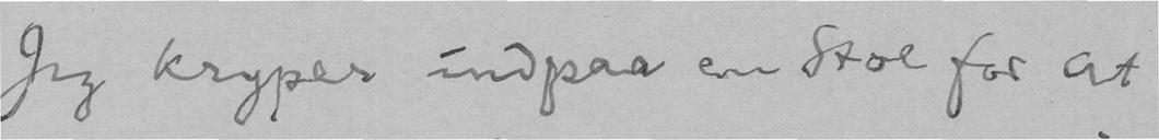Jeg kryper indpaa en Stol for at
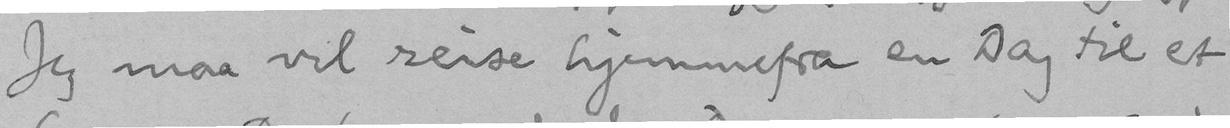Jeg maa vel reise hjemmefra en Dag til et
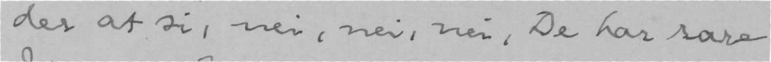der at si, nei, nei, nei, De har rare
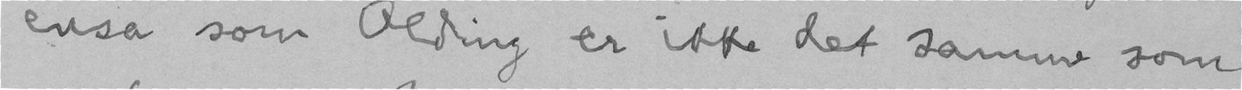ensa som Olding er ikke det samme som
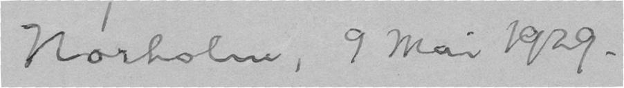Nørholm, 9 Mai 1929.
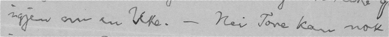igjen om en Uke. - Nei Tore kan nok
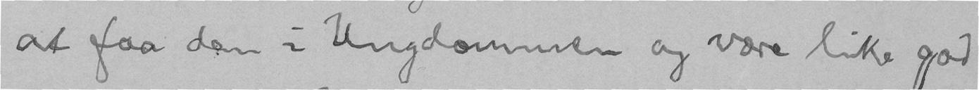at faa den i Ungdommen og være like god
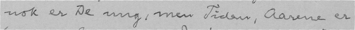nok er De ung, men Tiden, Aarene er
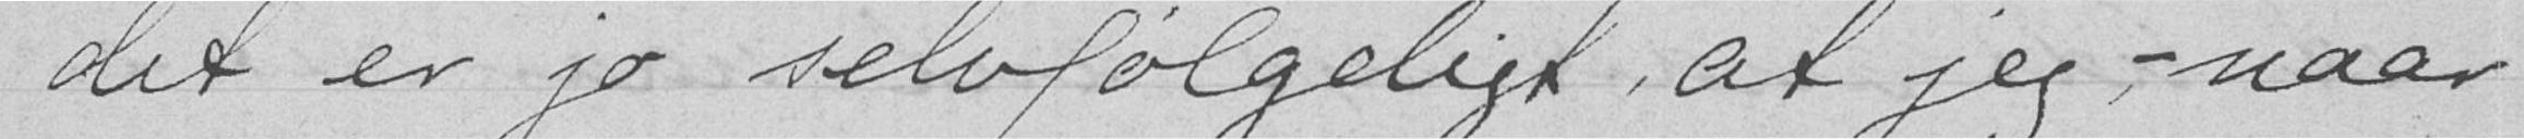det er jo selvfølgeligt, at jeg- naar
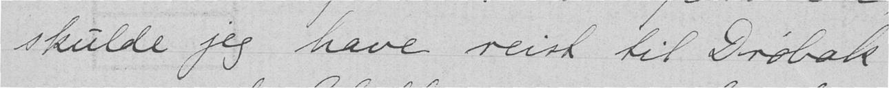skulde jeg have reist til Drøbak
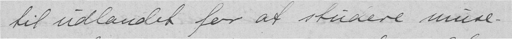til udlandet for at studere muse-
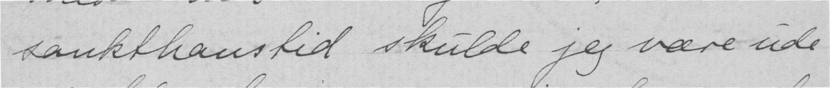sankthanstid skulde jeg være ude
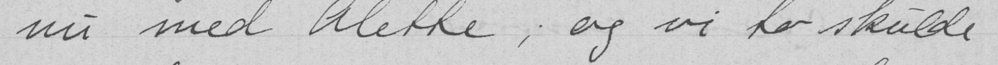nu med Alette, og vi to skulde
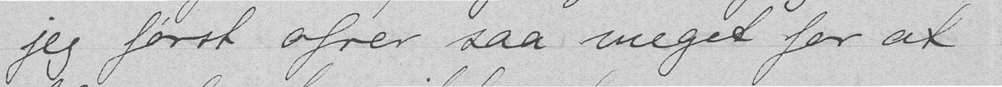jeg først ofrer saa meget for at
